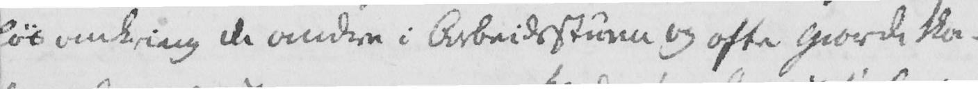løs omkring de andre i Arbeidsstuen og ofte giorde ska-
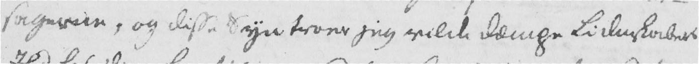sagerne, og disse Syn troer jeg vilde dæmpe Lidenskabers
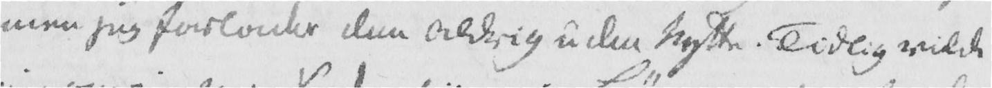men jeg forlader dem aldrig uden Nytte. Tidlig vilde
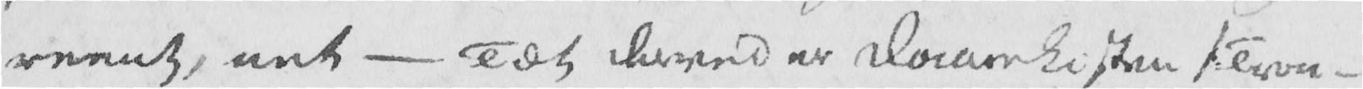reent, net - Tæt derved er Daarekisten /: Tron-
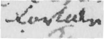Fortale
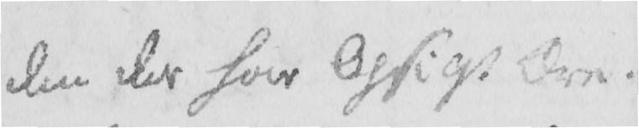den der har Opsigt Ære.
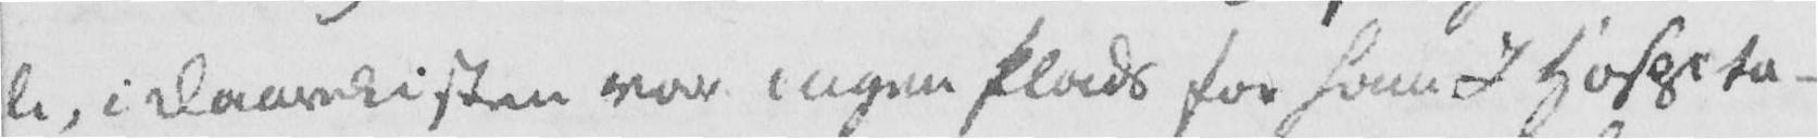de, i Daarekisten var ingen Plads for ham. I Hospita-
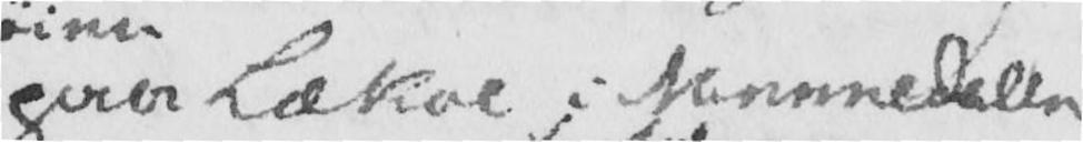mærkværdige Tildragelse paa Lækoe i Numedalen
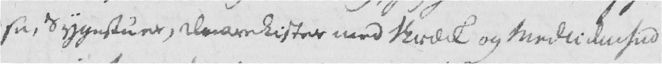se, Sygestuer, Daarekister med skræk og Medlidenhed
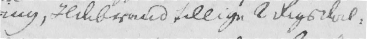ing, Ildebrand tillige 2 Rigsdal.
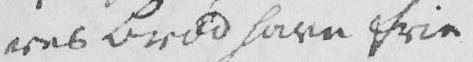res Brød have frie
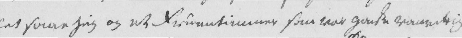let saae jeg og et Fruentimmer som var gandske vanvittig.
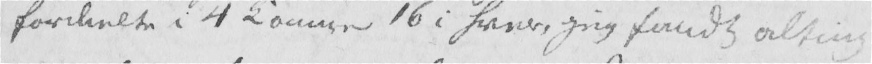fordelte i 4 Kammer 16 i hver, jeg fandt alting
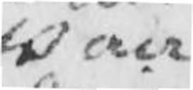Vaa
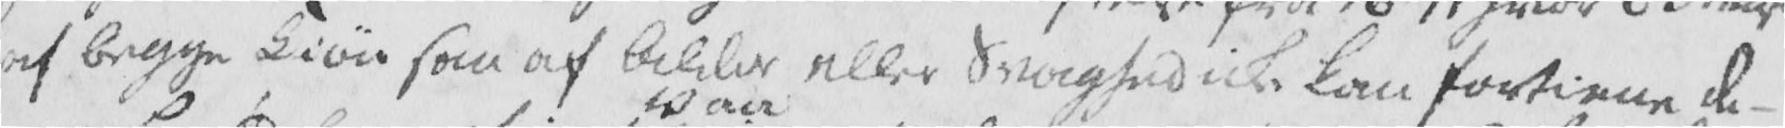af begge Kiøn som af Alder eller Svaghed icke kan fortiene de-
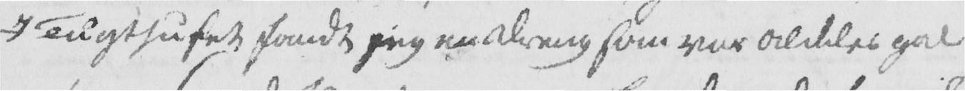I Tugthuset fandt jeg en Dreng som var aldeles gal
